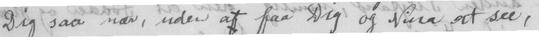Dig saa nær, uden at faa Dig og Nina at see,
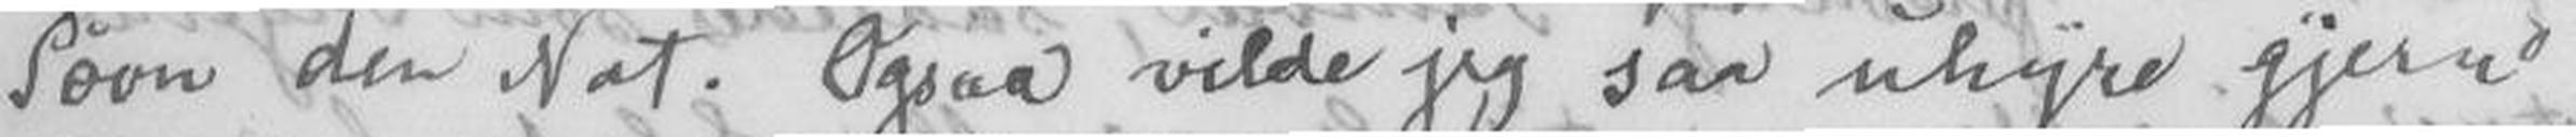Søvn den Nat. Ogsaa vilde jeg saa uhyre gjerne
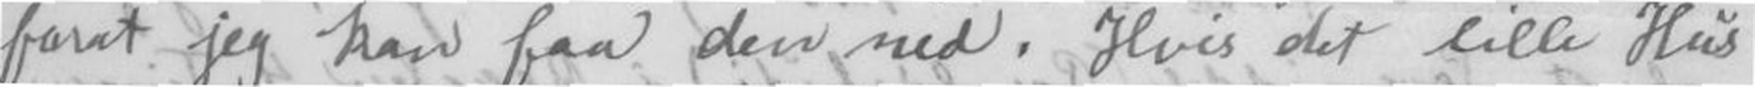forat jeg kan faa den ned. Hvis det lille Hus
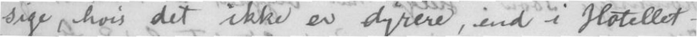sige, hvis det ikke er dyrere, end i Hotellet -
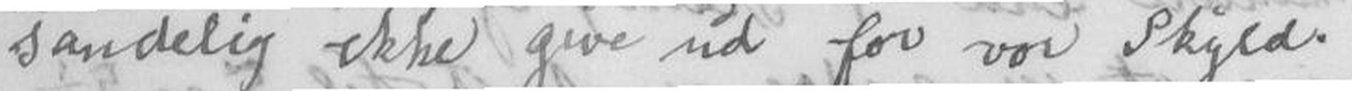sandelig ikke give ud for vor Skyld.
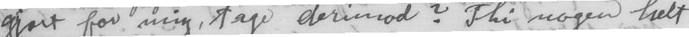gjort for mig, tage derimod ? Thi nogen helt
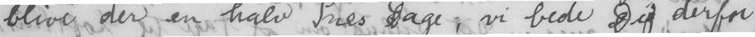blive der en halv Snes Dage; vi bede Dig derfor
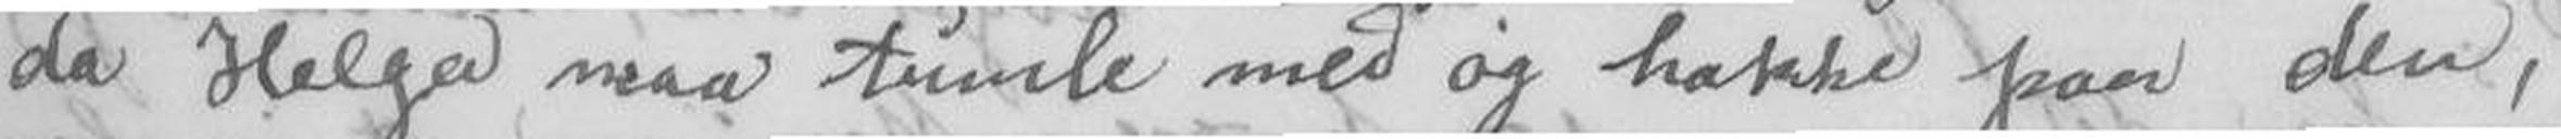da Helga maa tumle med og hakke paa den,
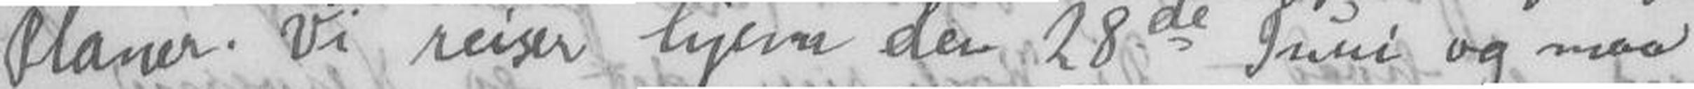Planer. Vi reiser hjem den 28de Juni og maa
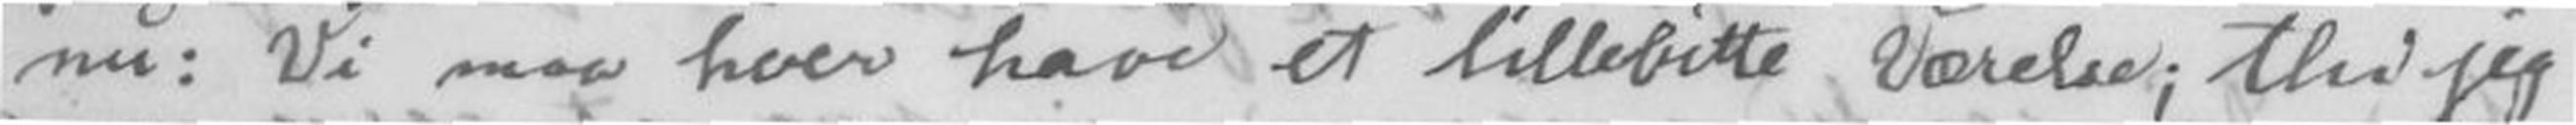nu: Vi maa hver have et lillebitte Værelse; thi jeg
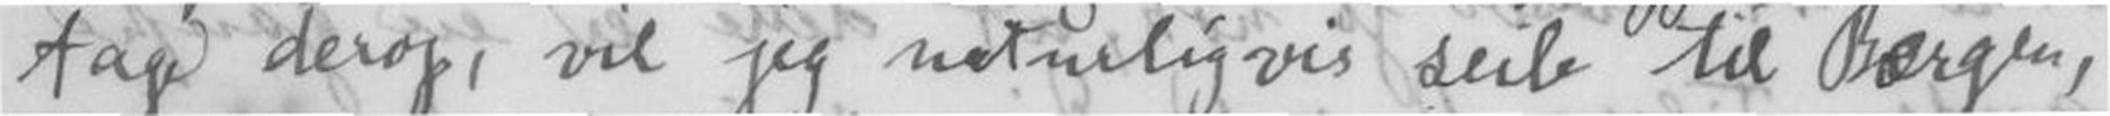tage derop, vil jeg naturligvis seile til Bergen,
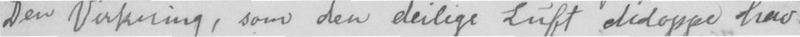Den Virkning, som den deilige Luft didoppe hav-
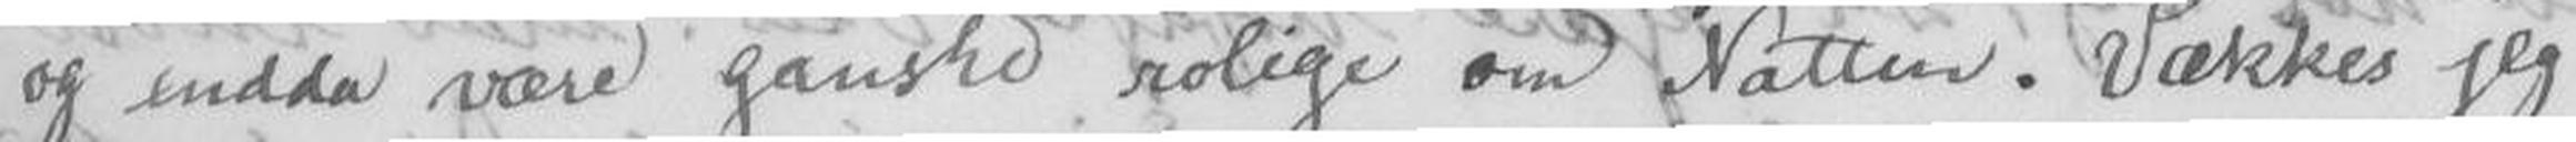og endda være ganske rolige om Natten. Vækkes jeg
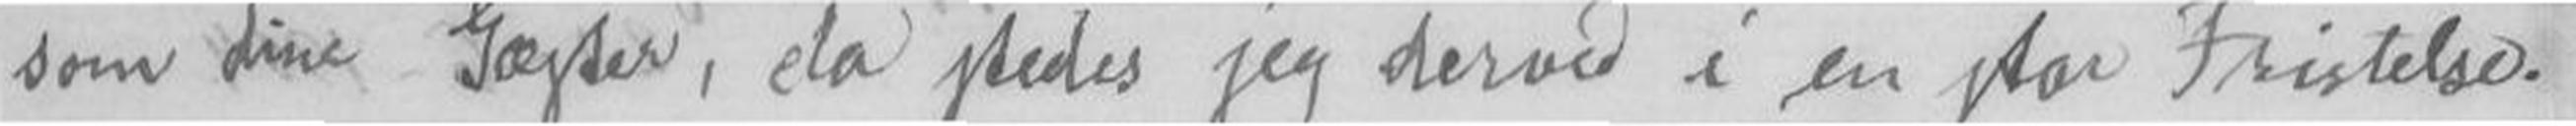som dine Gæster, da stedes jeg derved i en stor Fristelse.
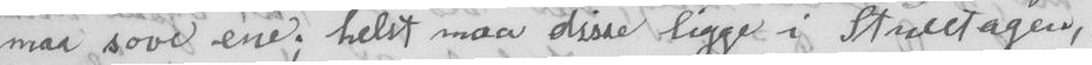maa sove ene; helst maa disse ligge i Stueetagen,
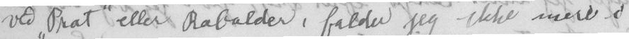ved Prat eller Rabalder, falder jeg ikke mere ik
